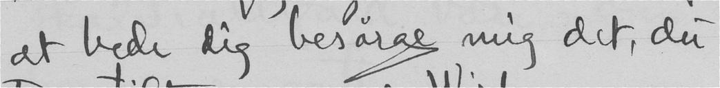at bede dig besørge mig det, du
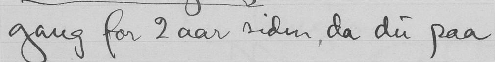gang for 2 aar siden, da du paa
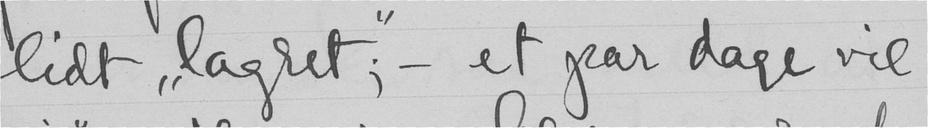lidt "lagret"; - et par dage vil
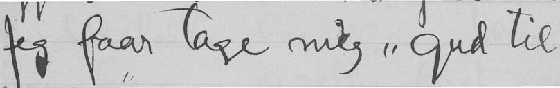Jeg faar tage mig "gud til
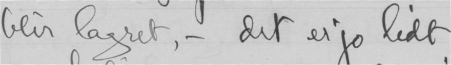blir lagret, - det er jo lidt
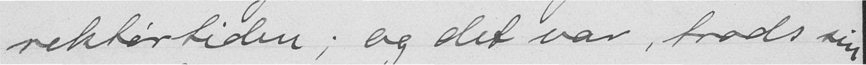rektørtiden; og det var, trods sin
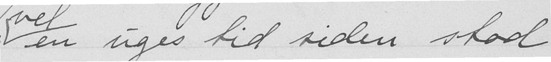en uges tid siden stod
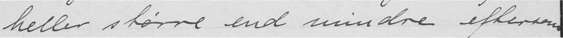heller større end mindre eftersom
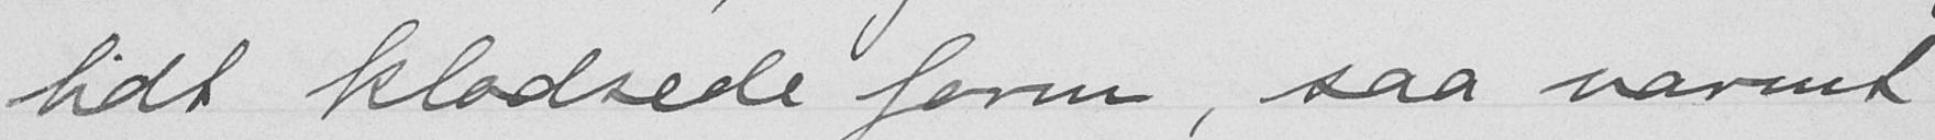lidt klodsede form, saa varmt
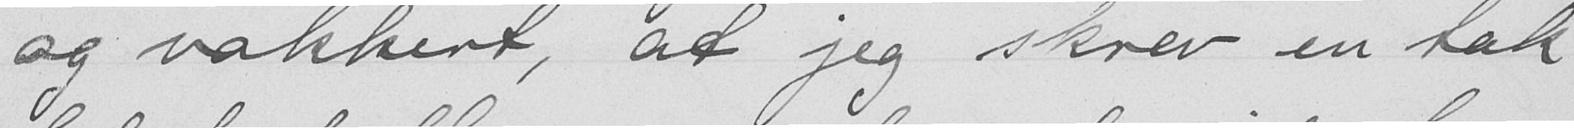og vakkert, at jeg skrev en tak
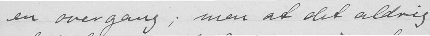en overgang; men at det aldrig
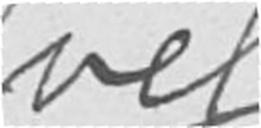vel
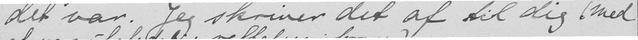det var. Jeg skriver det af til dig (med
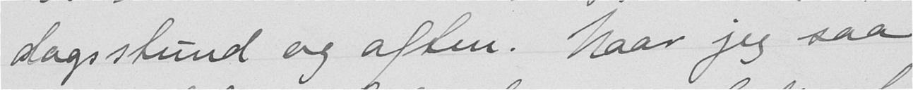dagsstund og aften. Naar jeg saa
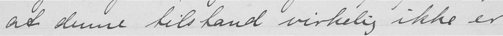at denne tilstand virkelig ikke er
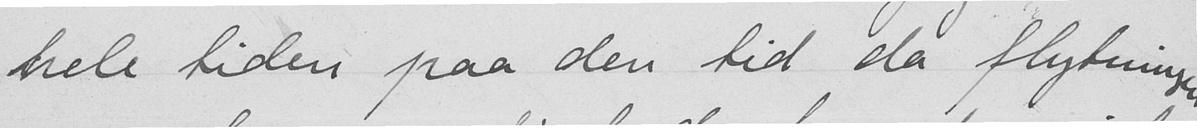hele tiden paa den tid da flytningen
